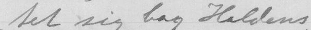tet sig bag Haldens
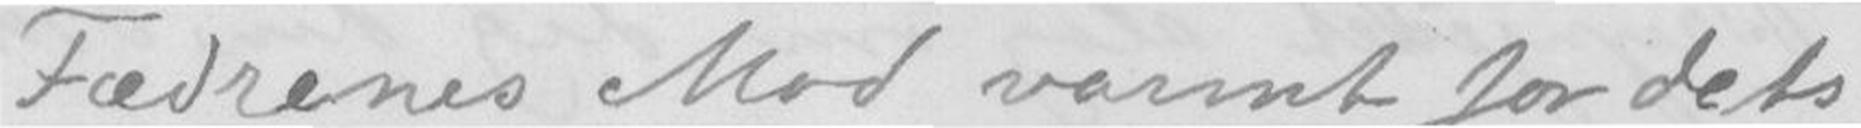Fædrenes Mod varmt for dets
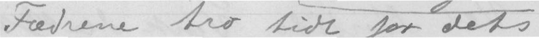Fædrene tro tidt for dets
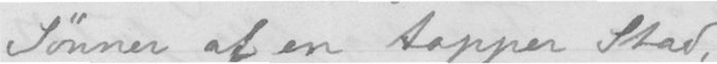Sønner af en tapper Stad,
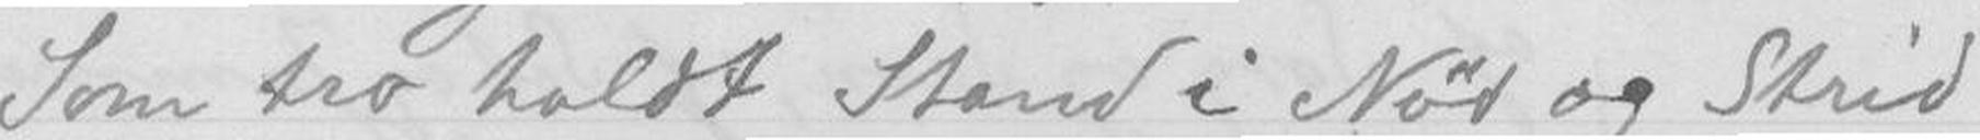Som tro holdt Stand i Nød og strid
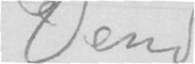(Vend!)
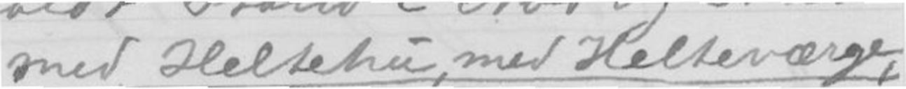med Heltetru, med Helteværge,
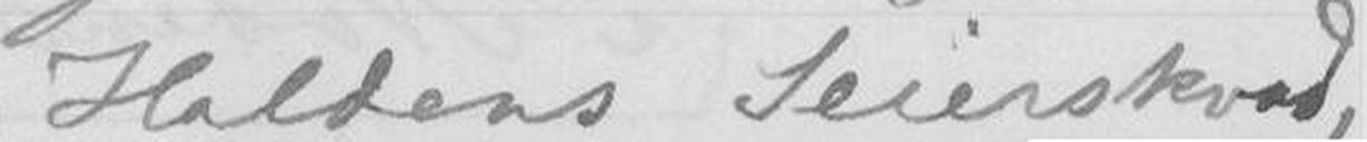Haldens Seierskvad!
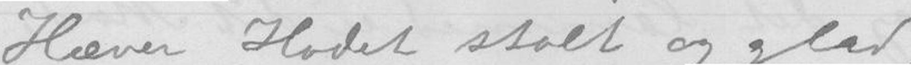Hæver Hodet stolt og glad,
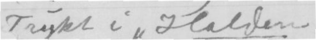Trygt i "Halden"
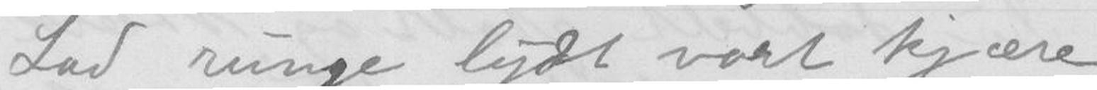Lad runge lydt vort kjære
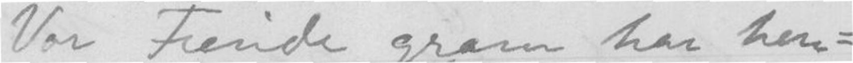Vor Fiende gram har hen-
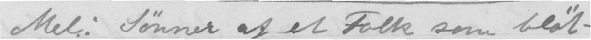Mel: Sønner af et Folk som bløt -
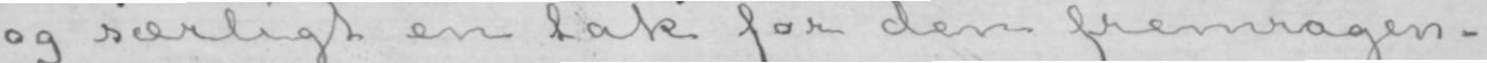og særligt en tak for den fremragen-
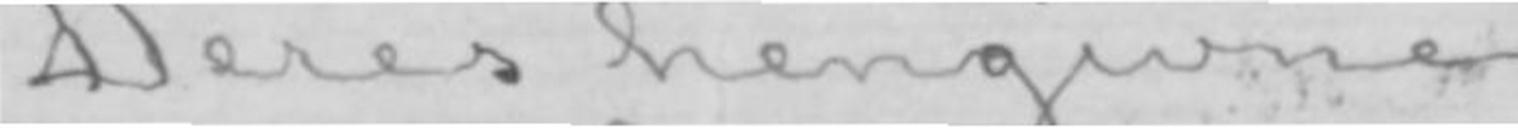Deres hengivne
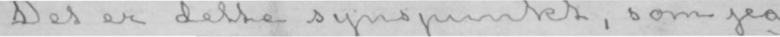Det er dette synspunkt, som jeg
